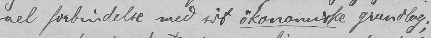nel forbindelse med sit økonomiske grundlag;
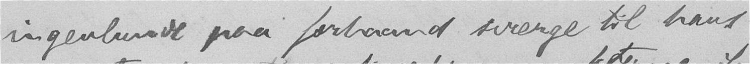ingenlunde paa forhaand sværge til hans
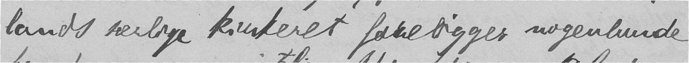lands særlige kirkeret foreligger nogenlunde
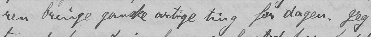ren bringe ganske artige ting for dagen. Jeg
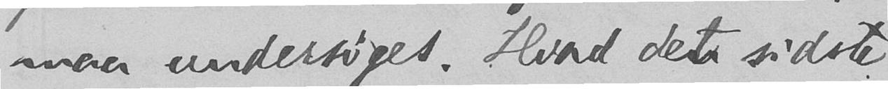maa undersøges. Hvad det sidste
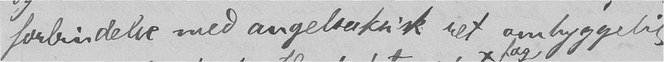forbindelse med angelsaksisk ret omhyggelig
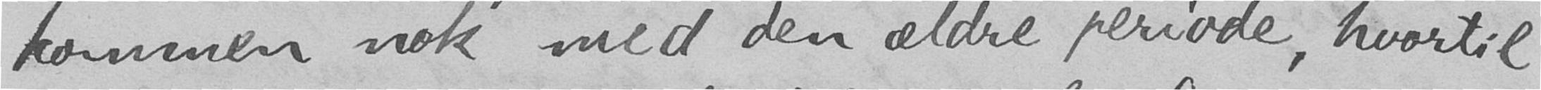kommen nok med den ældre periode, hvortil
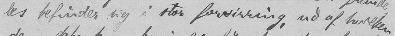les befinder sig i stor forvirring, ud af hvilken
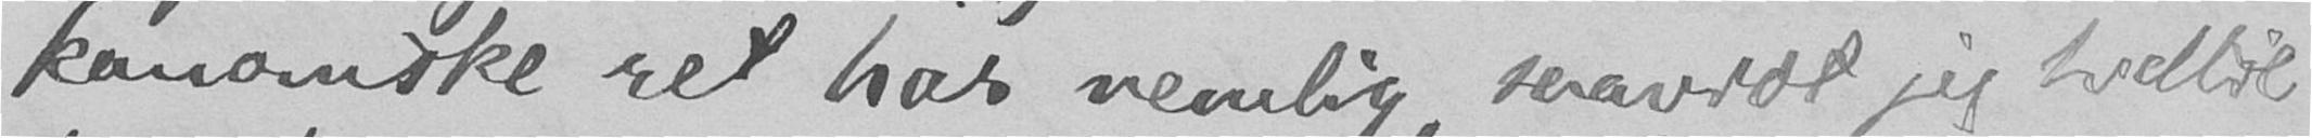kanoniske ret har nemlig, saavidt jeg hidtil
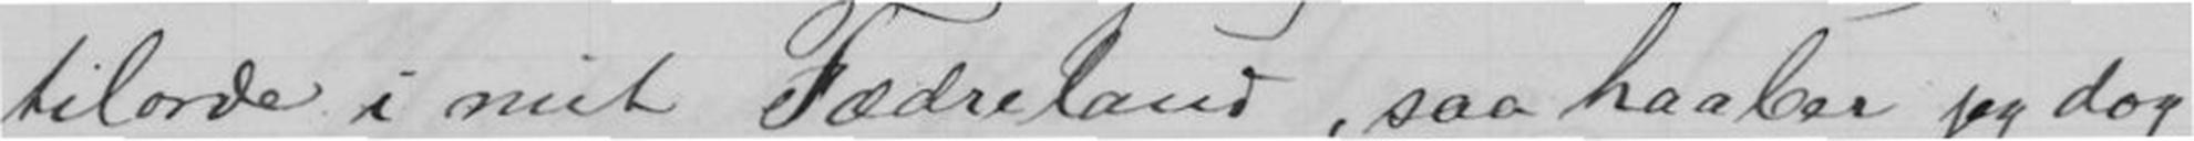tilorde i mit Fædreland, saa haaber jeg dog
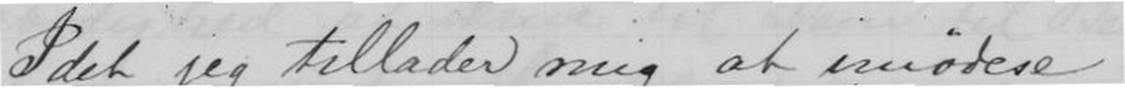Idet jeg tillader mig at imødese
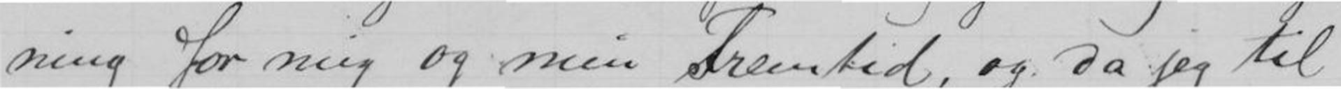ning for mig og min Fremtid, og da jeg til
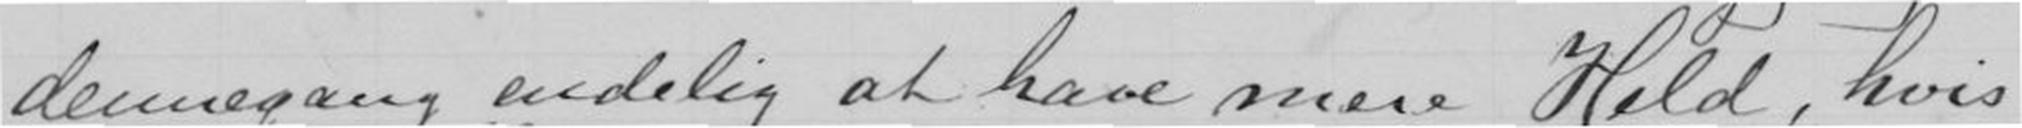dennegang endelig at have mere Held, hvis
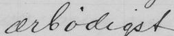ærbødigst
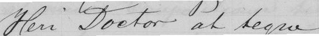Herr Doctor at tegne
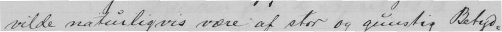vilde naturligvis være at stor og gunstig Betyd-
